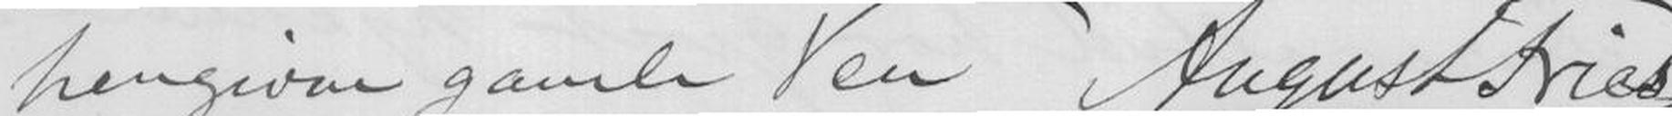hengivne gamle Ven August Fries
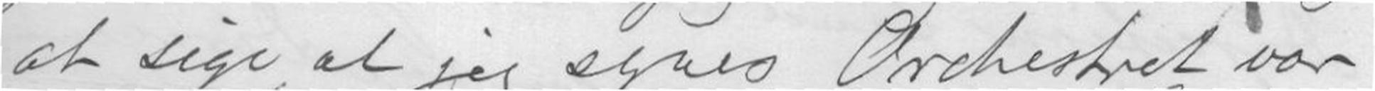at sige, at jeg synes Orchestret var
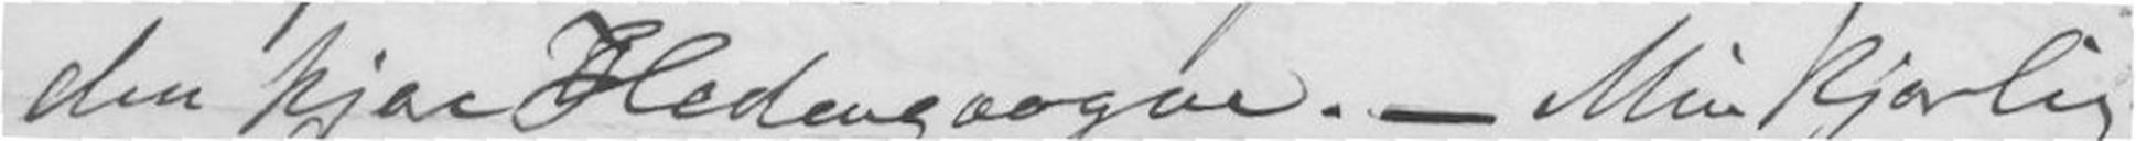den Hedengangen. - Min kjærlig Hilsen
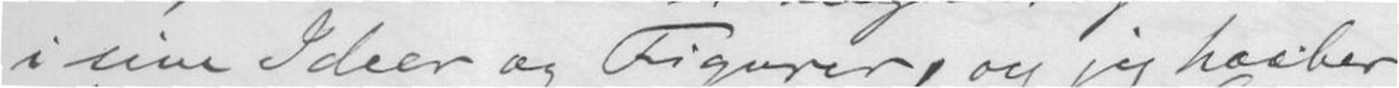i sine Ideer og Figurer, og jeg haaber
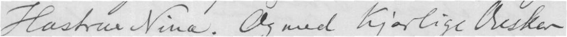Hustru Nina. Og med kjærlige Ønsker
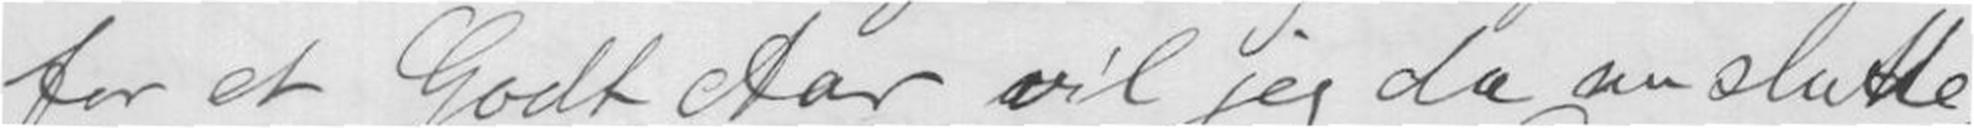for et Godt Aar vil jeg da nu slutte.
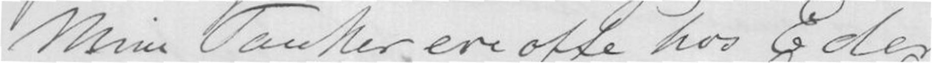Mine Tanker ere ofte hos Eder
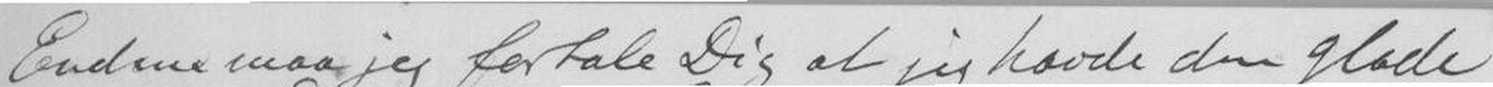Endnu maa jeg fortæle Dig at jeg havde den glæde
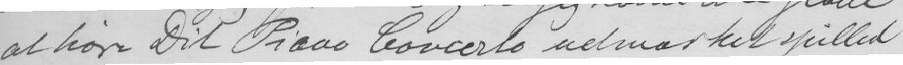at høre Dit Piano Concerte udmærket spilled
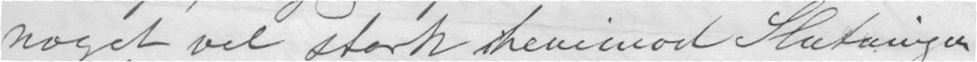noget vel stark henimod Slutningen
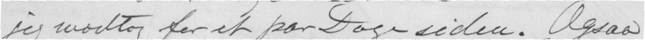jeg modtog for et par Dage siden. Ogsaa
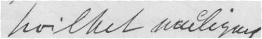hvilket muligent
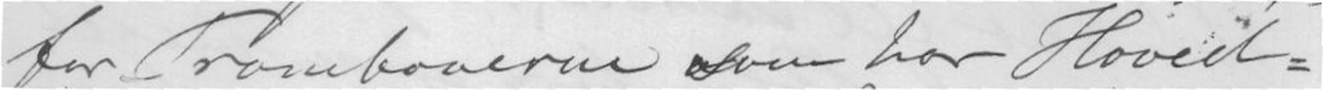for Trombonerne som har Hoved-
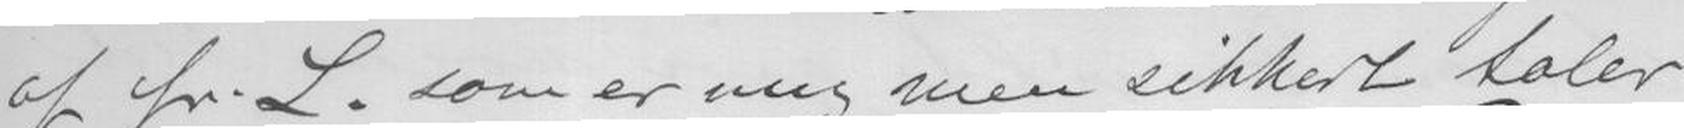af hr. L. som er ung men sikkert taler
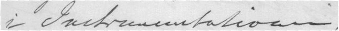i Instrumentationen
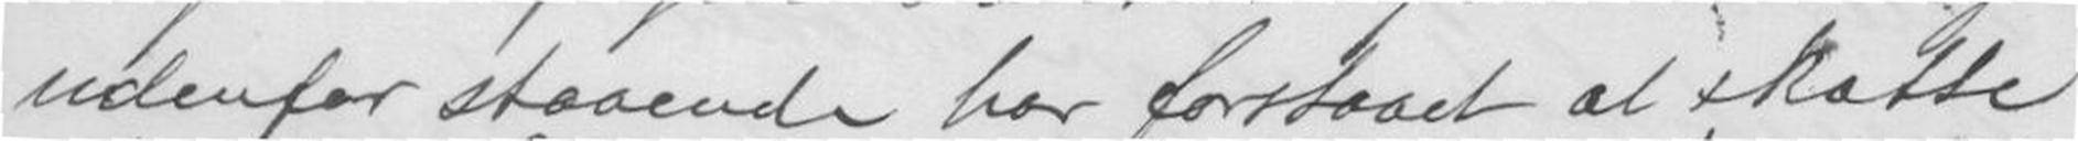udenfor staaende har forstaaet at skatte
